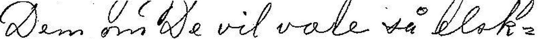Dem, om De vil være så elsk-
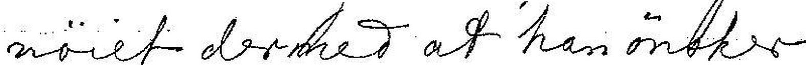nøiet dermed, at han ønsker
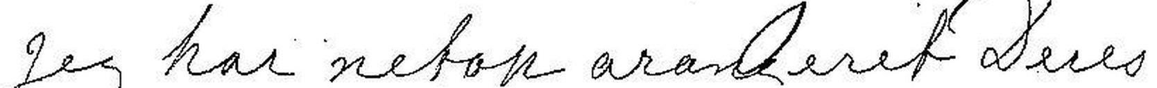Jeg har netop arangeret Deres
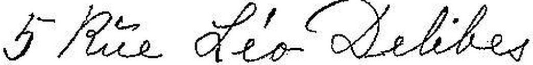5 Rue Léo Delibes
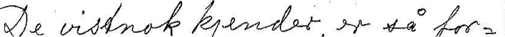De vistnok kjender, er så for-
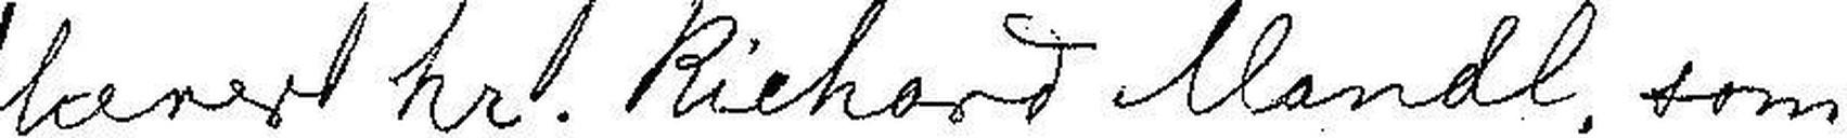lærer hr. Richerd Mandl, som
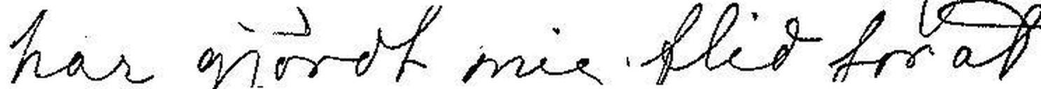har gjort mig flid for at
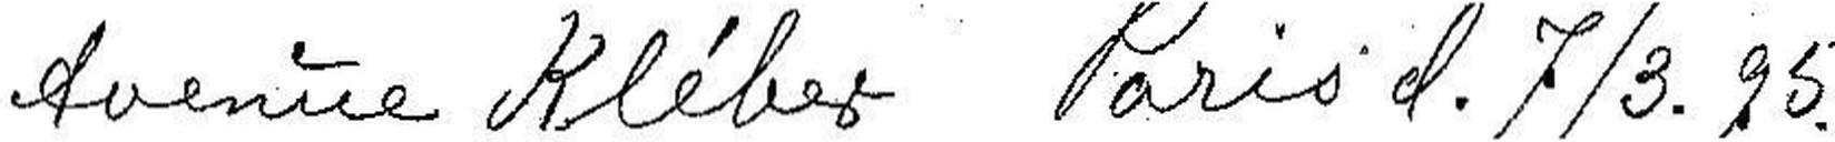Avenue Kléber Paris d. 7/3. 95.
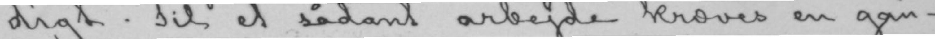digt. Til et sådant arbejde kræves en gan-
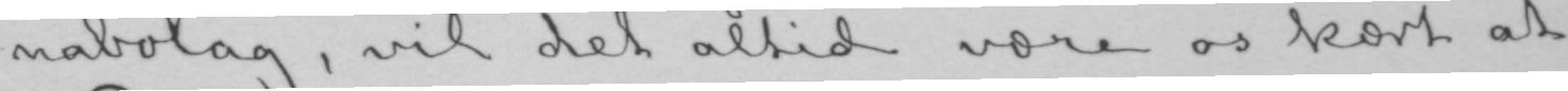nabolag, vil det altid være os kært at
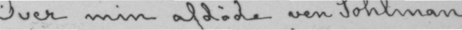Over min afdøde ven Sohlman
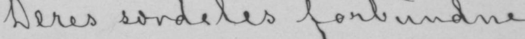Deres særdeles forbundne
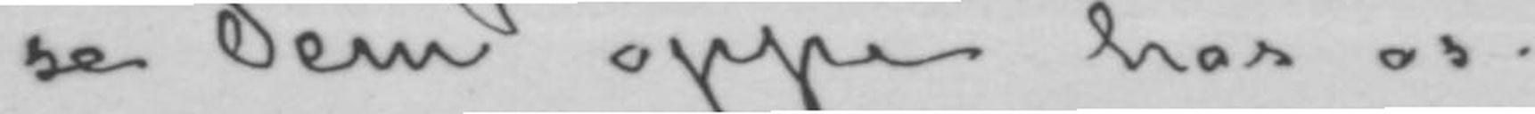se Dem oppe hos os.
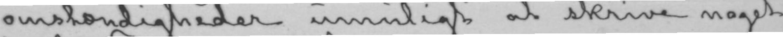omstændigheder umuligt at skrive noget
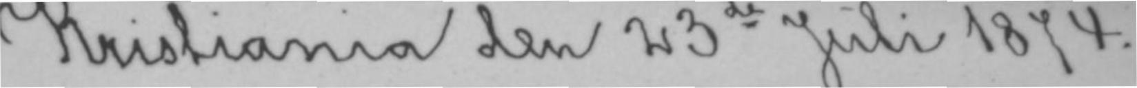Kristiania den 23de Juli 1874.
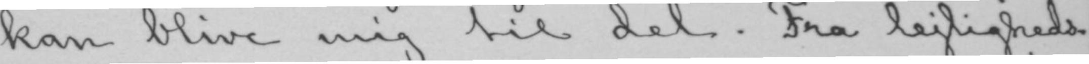kan blive mig til del. Fra lejligheds-
